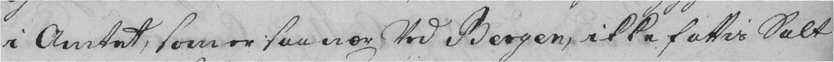i Amtet, som er saa nær ved Bergen, ikke fattis Salt
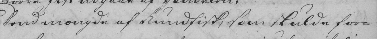Dend mængde af Rundfisk, som skulde for-
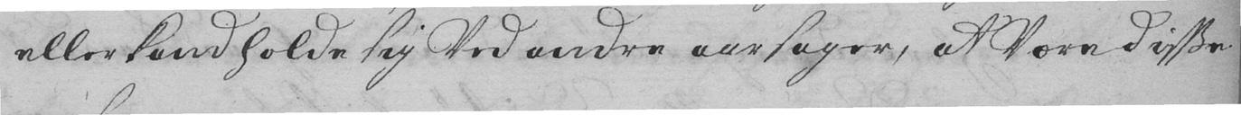eller kand holde sig ved andre aarsager, at Være disse
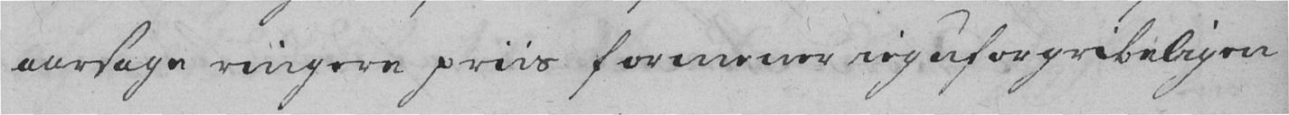aarsage ringere priis formener ieg uforgribeligen
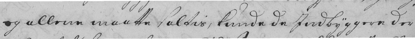og allene maatte saltis, kunde de Indbyggere der
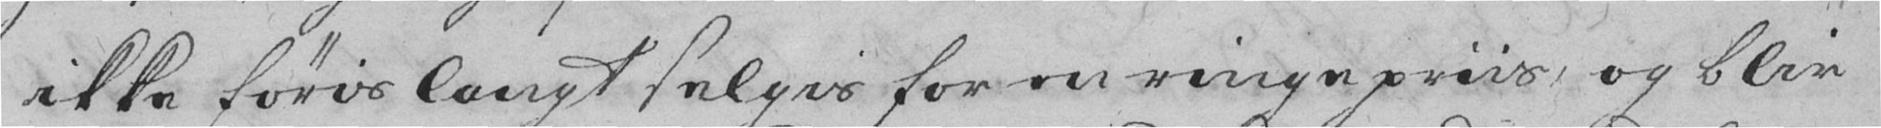ikke føris langt selgis for en ringe priis, og blir
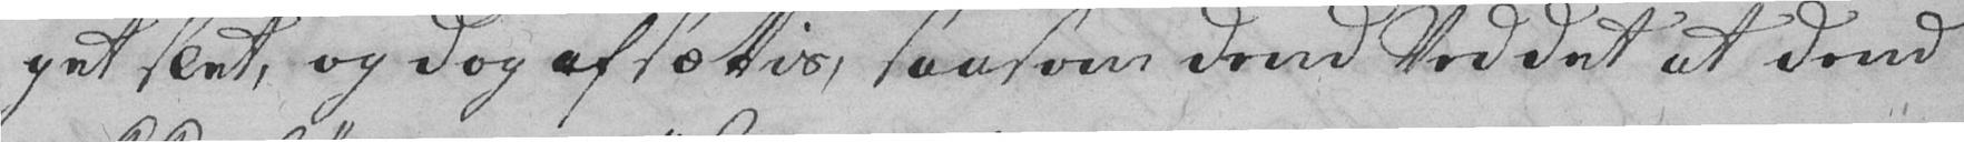get slet, og dog afsættis, saasom dend Ved det at dend
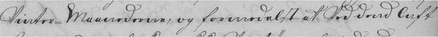Vinter-Maanederne, og formedelst at Ved dend luft
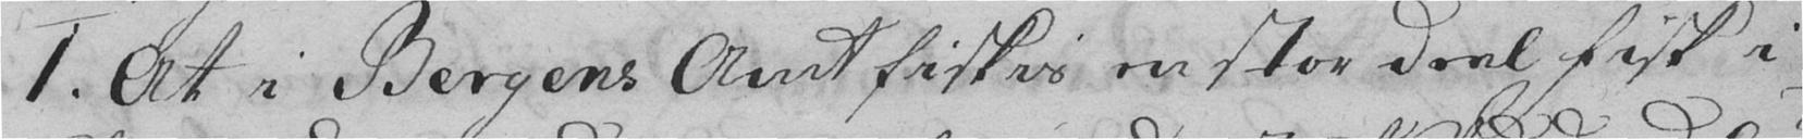1. At i Bergens Amt fiskis en stor deel Fisk i
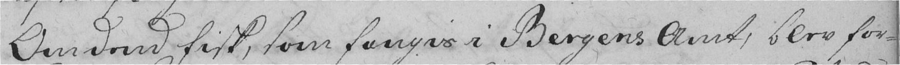Om dend Fisk, som fangis i Bergens Amt, blev for-
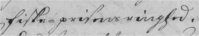Fiske-prisens ringhed.
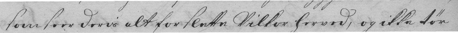som seer deris alt for slette Vilkor herved, og ikke tør
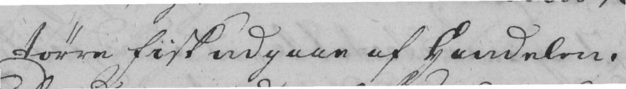tørre Fisk udgaae af Handelen.
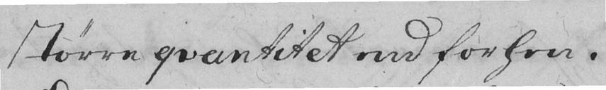større qvantitet end forhen.
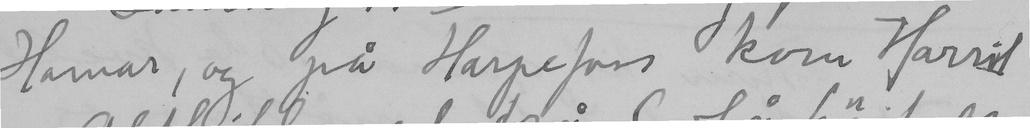Hamar, og på Harpefoss kom Harriet
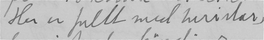Her er fullt med turistar,
